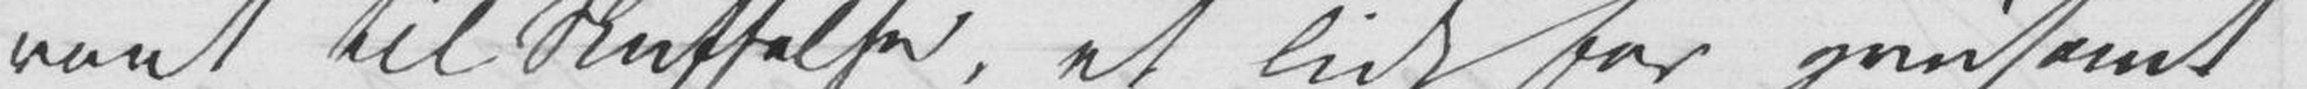vant til Skuffelser, et lidt for grusomt
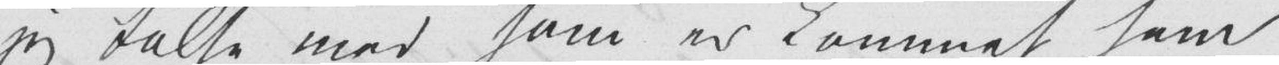jeg talte med ham er kommet ham
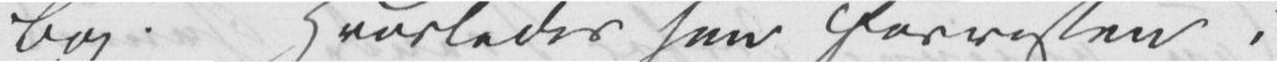Bog. Hvorledes han forresten i
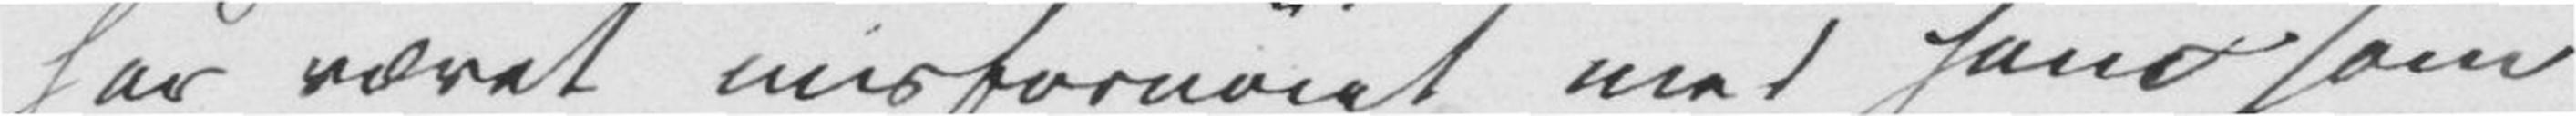har været misfornøiet med ham
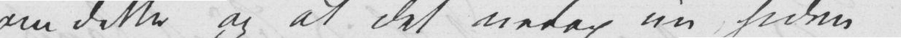om dette og at det netop nu siden
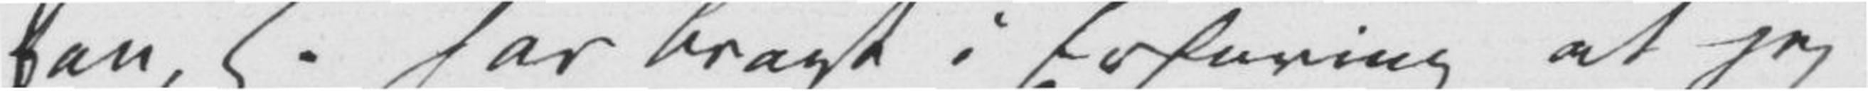han, H. har bragt i Erfaring at jeg
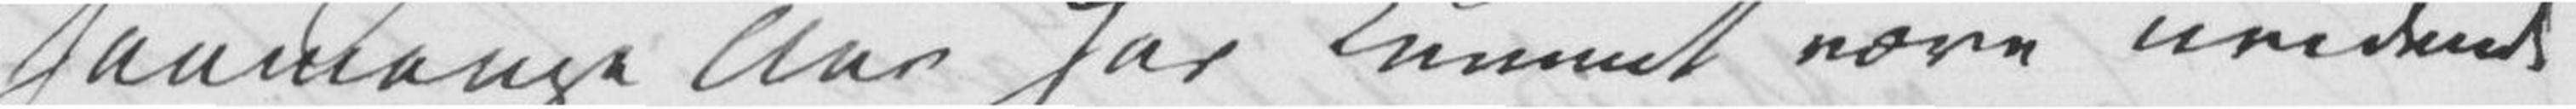saamange Aar har kunnet være uvidende
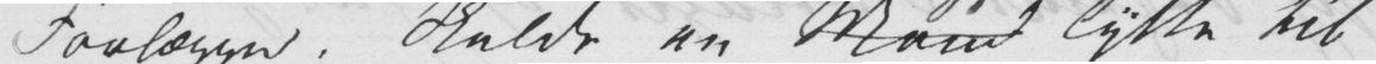som Forlægger. Skulde en Mand lytte til
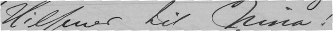Hilsener til Nina!
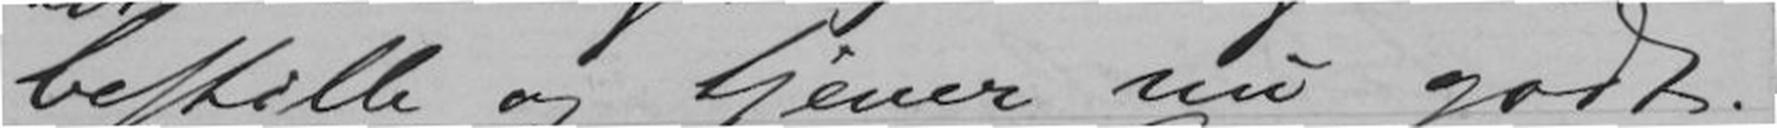bestille og tjener nu godt.
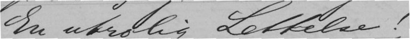En utrolig Lettelse!
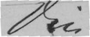Din
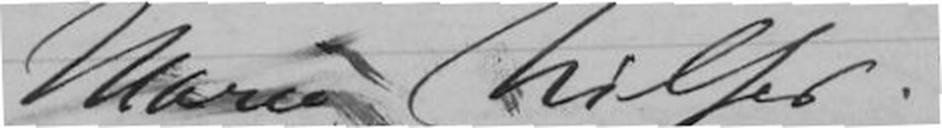Marie Hilser!
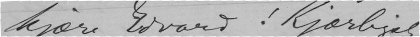kjære Edvard! Kjærligste
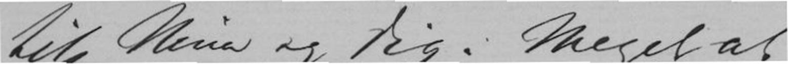til Nina og Dig. Meget at
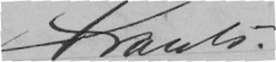Frants
